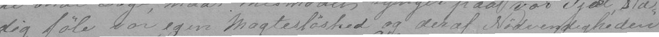dig føle vor egen Magtesløshed og deraf Nødvendigheden
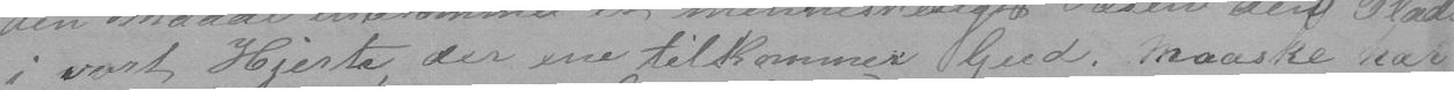i vort Hjerte, der ene tilkommer Gud. Maaske har
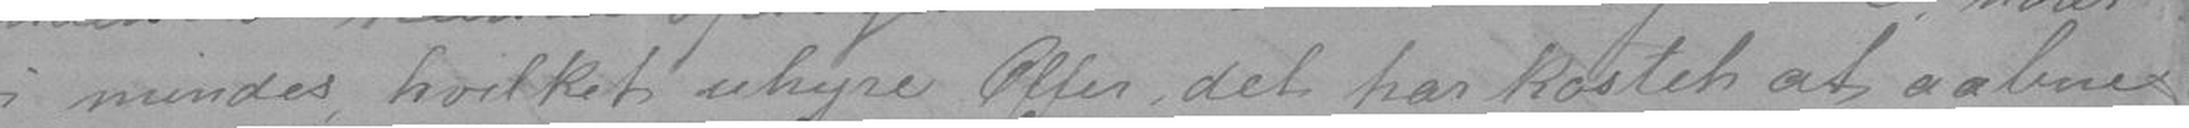vi mindes hvilket uhyre Offer det har kostet at aabne
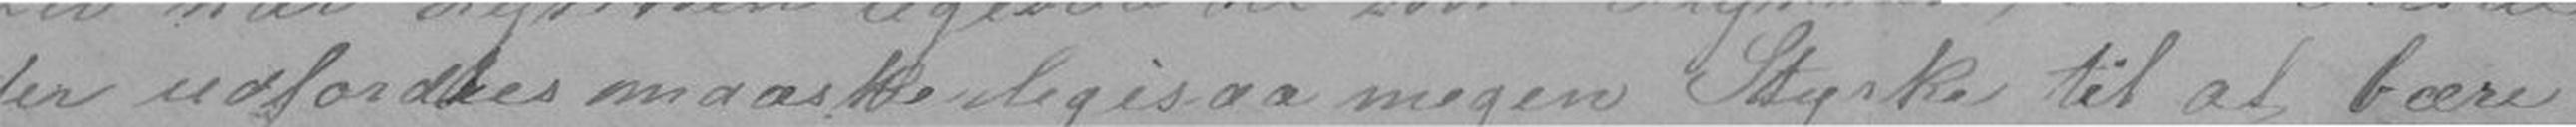der udfordres maaske ligesaa megen Styrke til at bære
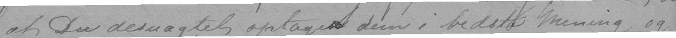at Du desuagtet optager dem i bedste Mening og
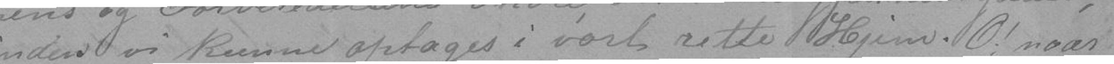inden vi kunne optages i vort rette Hjem. O! naar
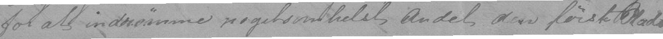os godt for at indrømme nogetsomhelst Andet den første Plads
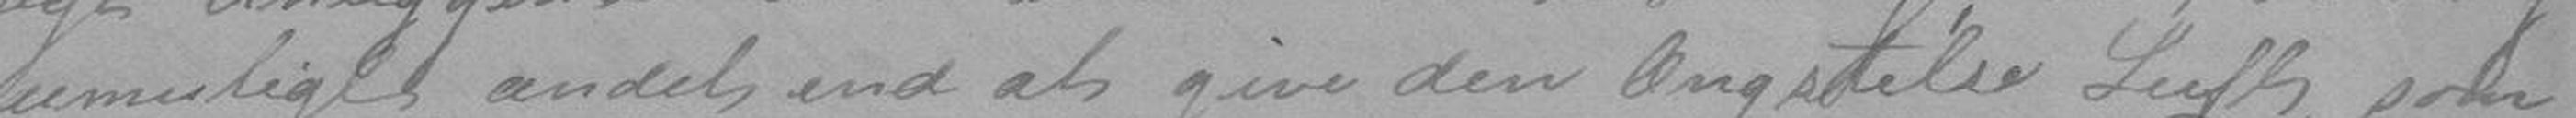umuligt andet end at give den Ængstelse Luft, som
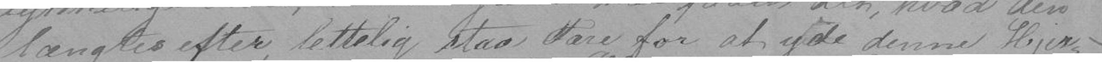længtes efter, lettelig staa Fare for at yde denne Hjer-
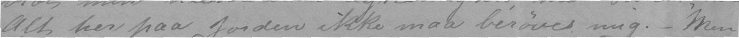Alt her paa Jorden ikke maa berøres mig. - Men
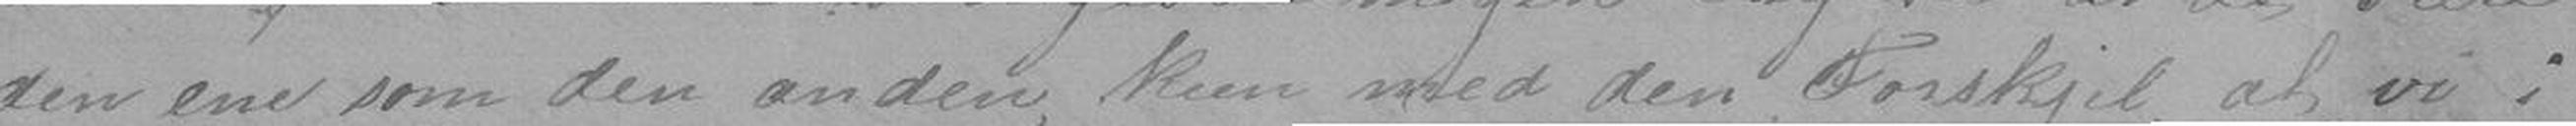den ene som den anden, kun med den Forskjel, at vi i
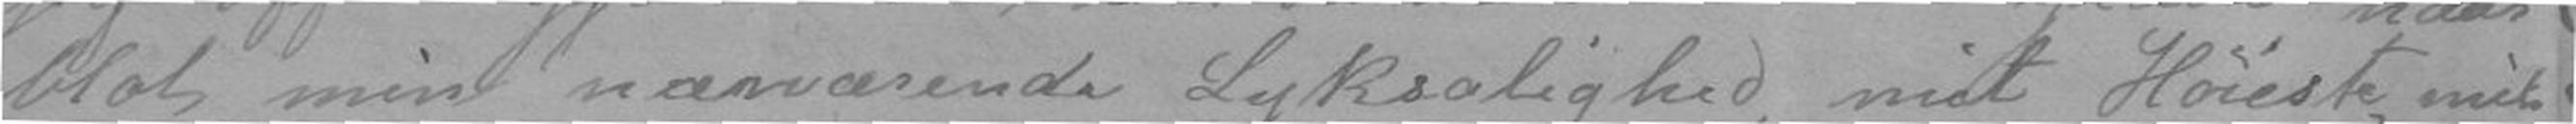blot min nærværende Lyksalighed, mit Høieste, mit
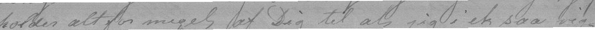holder altfor meget af Dig til at jeg i et saa vig-
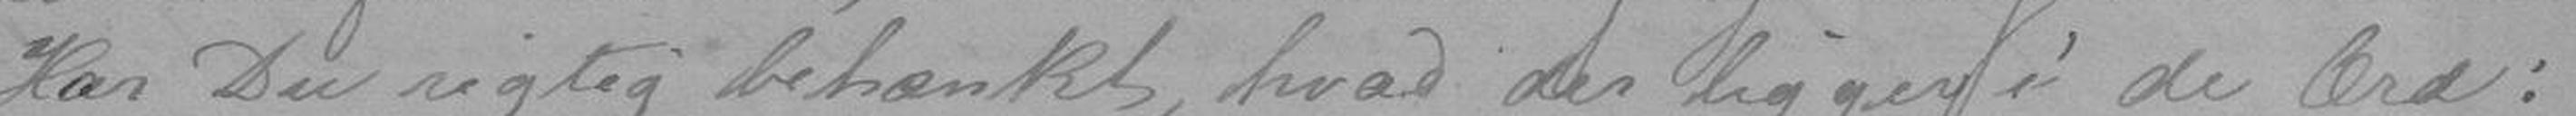Har Du rigtig betænkt, hvad der ligger i de Ord:
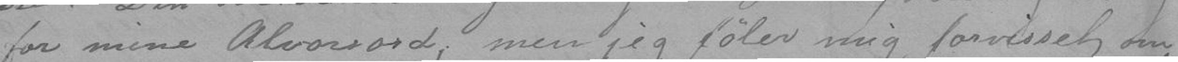for mina Alvorsord, men jeg føler mig forvisset om,
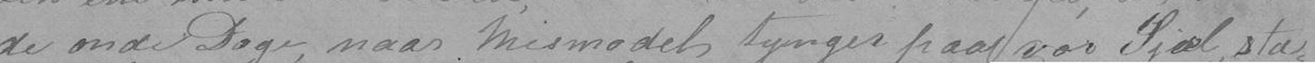de onde Dage, naar Mismodet tynges paa vor Sjæl, sta-
